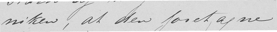niken, at den foretagne
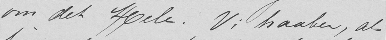om det Hele. Vi haaber, at
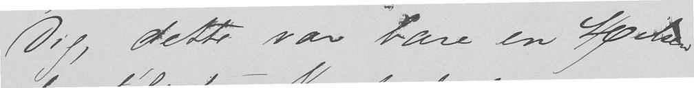Dig, dette var bare en Hilsen
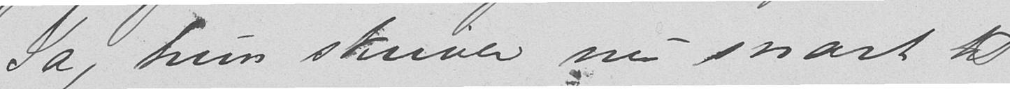Ja, hun skriver nu snart til
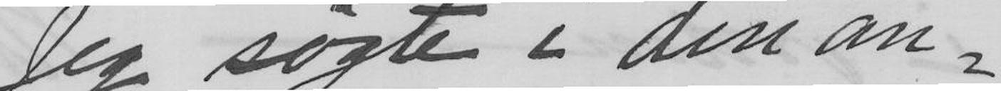Jeg søgte i den an-
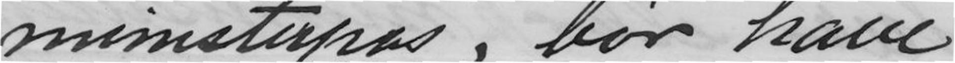ministerpas, bør have
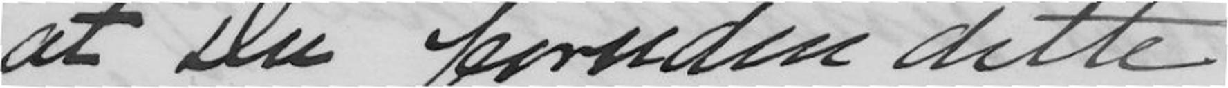at Du foruden dette
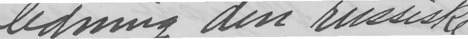ledning den russiske
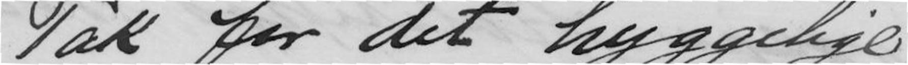Tak for det hyggelige
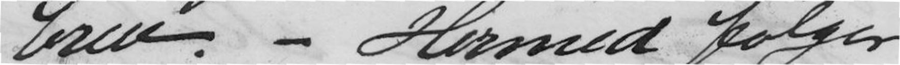brev. - Hermed følger
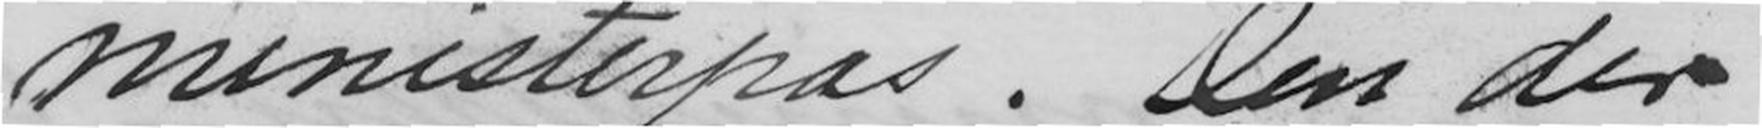ministerpas. Den der
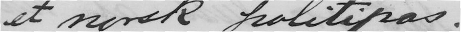et norsk politipas.
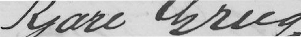Kjære Grieg
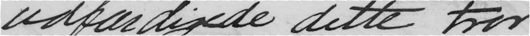udfærdigede dette tror,
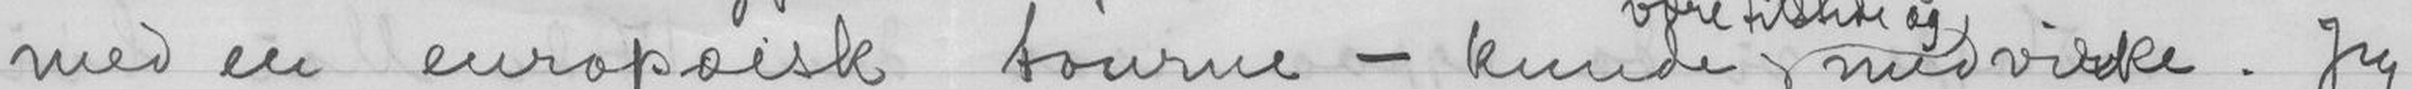med en europeisk tourne - kunde med virke. Jeg
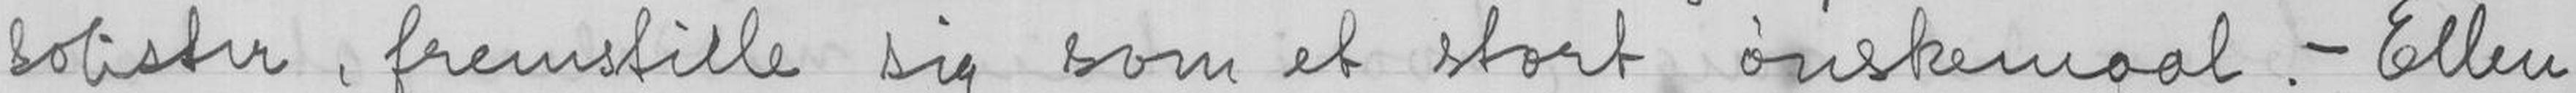solister, fremstille sig som et stort ønskemaal. - Ellen
